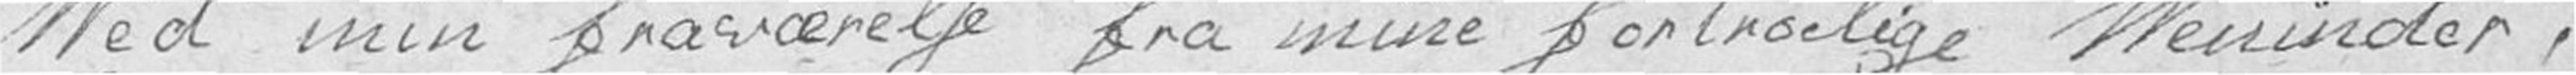Ved min fraværelse fra mine fortroelige Veninder,
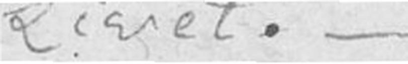Livet. –
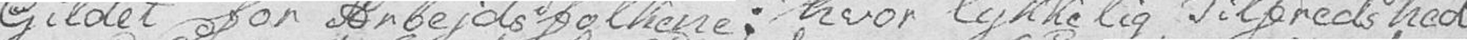Gildet for Arbejdsfolkene: hvor lykkelig Tilfredshed
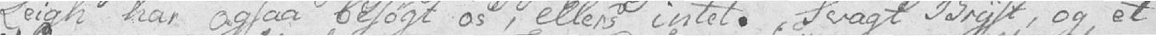Leigh har ogsaa besøgt os, ellers intet. Svagt Bryst, og et
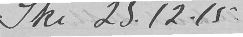Ski 23.12.15
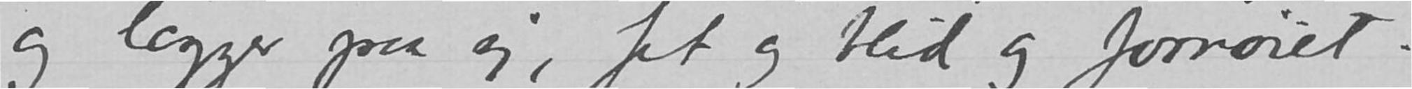og lægger paa sig, fet og blid og fornøiet
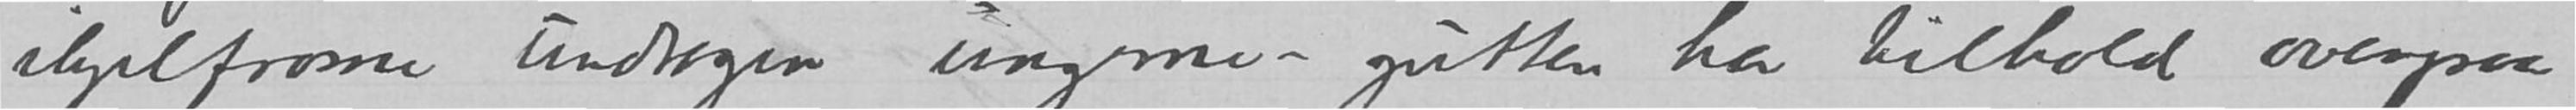ihjelfrosne undtagen ungerne – gutten har tilhold ovenpaa
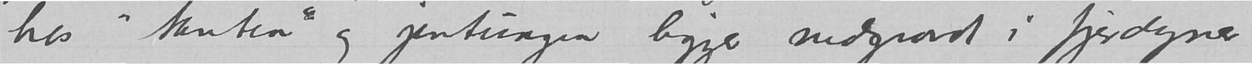hos «tanten» og jentungen ligger nedgravd i fjerdyner
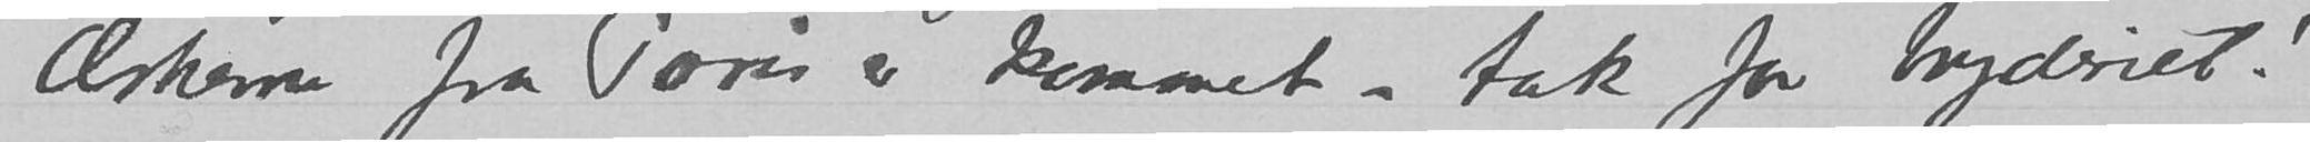Æskerne fra Paris er kommet – tak for bryderiet!
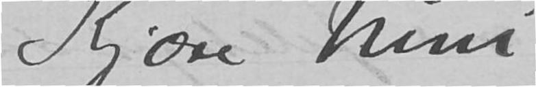Kjære Nini
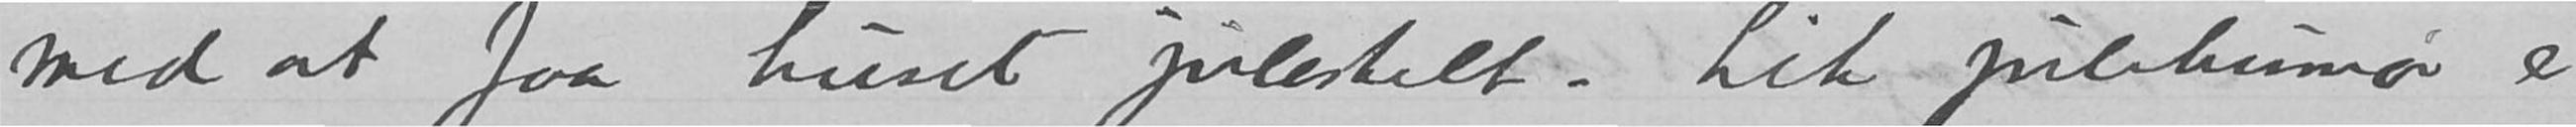med at faa huset julestelt – lite julehumør er
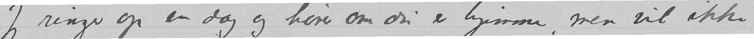Jeg ringer op en dag og hører om du er hjemme, men vil ikke
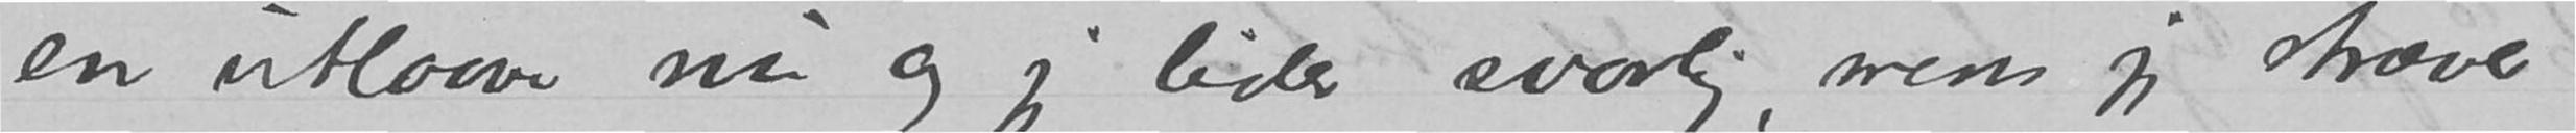en utlaave nu og jeg lider alvorlig, mens jeg stræver
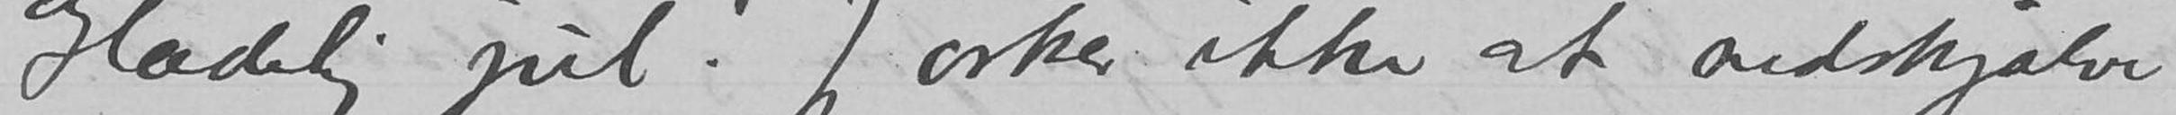Glædelig jul! Jeg orker ikke at nedskjælve
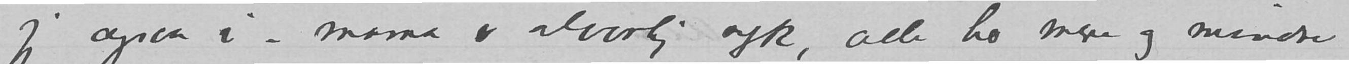jeg ogsaa i – mama er alvorlig syk, alle her mere og mindre
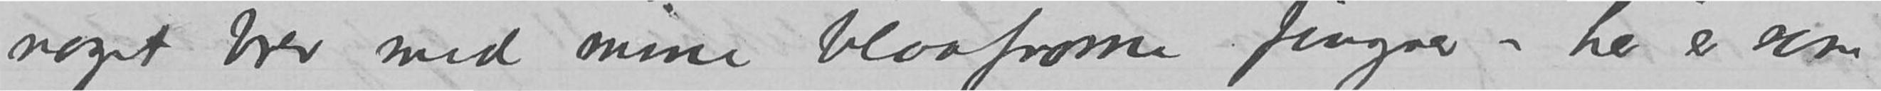noget brev med mine blaafrosne fingrer – her er
